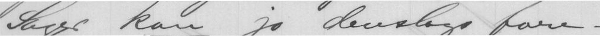Sager kan jo deslags fore-
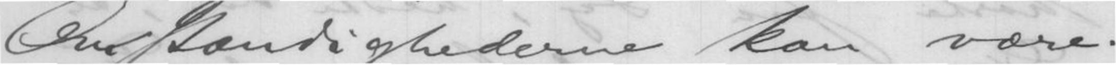Omstændighederne kan være.
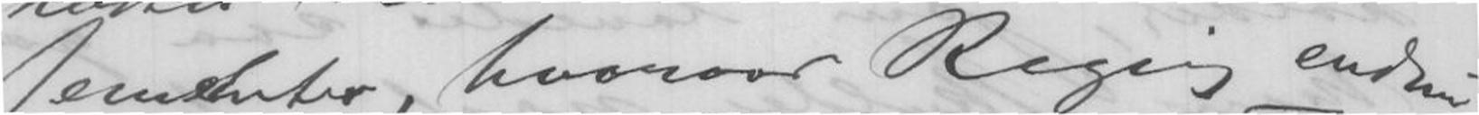sementer, hvorom Regning endnu
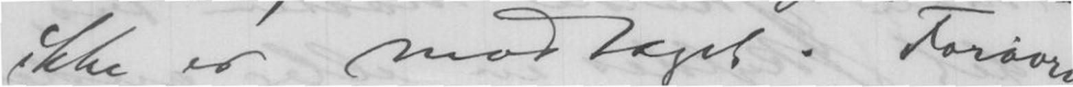ikke er modtaget. Forøvrigt
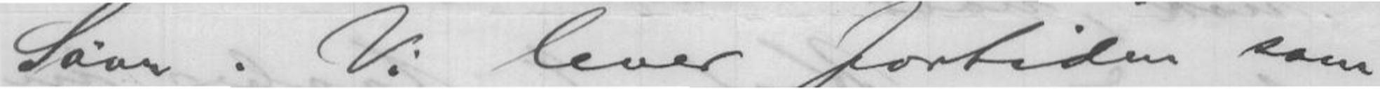Søvn. Vi lever fortiden som
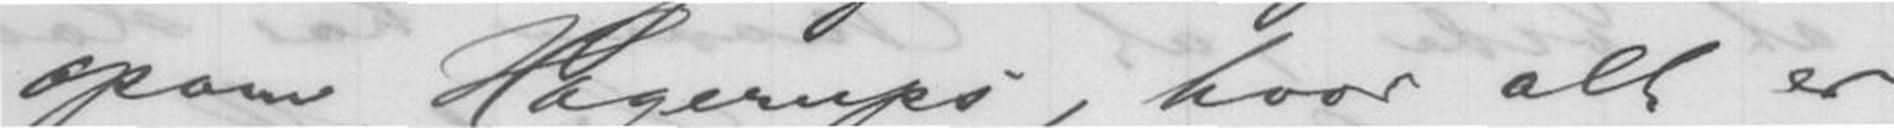opom Hagerups, hvor alt er
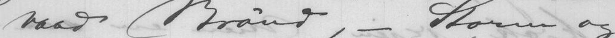vaad Brønd, - Storm og
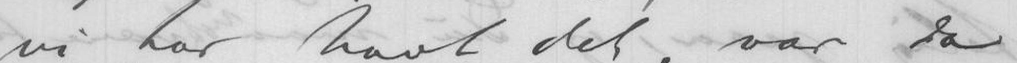vi har havt det, var da
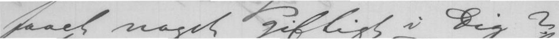faaet noget giftigt i Dig?
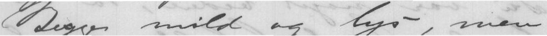Begge mild og lys, men
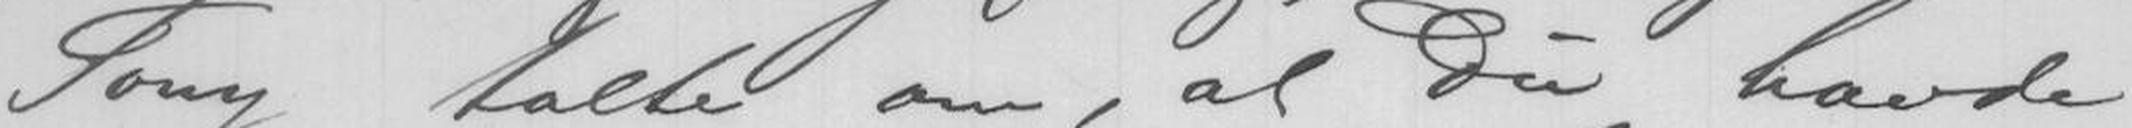Tony talte om, at Du havde
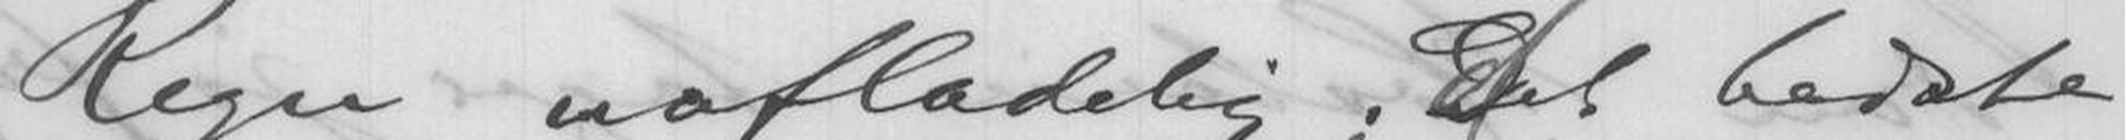Regn uafladelig; det bedste,
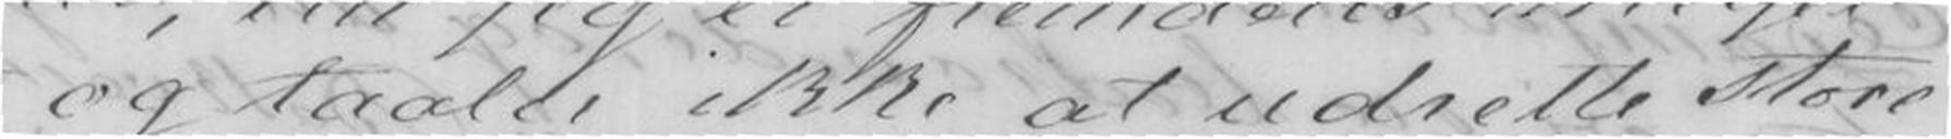og taale, ikke at udrette store
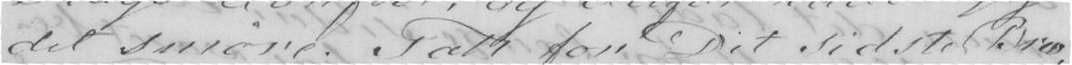det smøre. Tak for Dit sidste Brev,
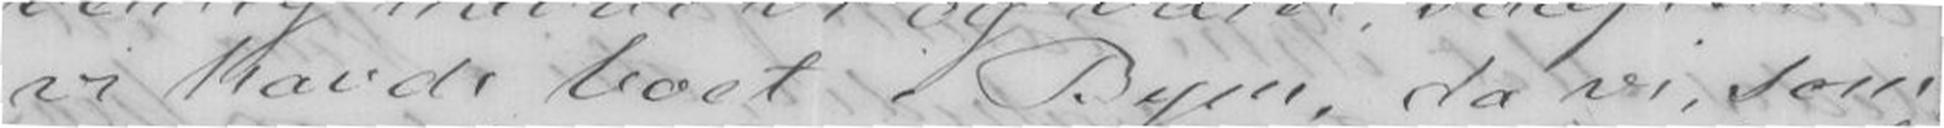i havde boet i Byen, da vi som
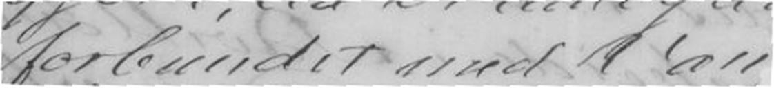forbundet med Van
